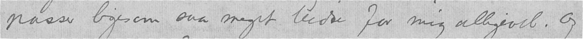passer ligesom saa meget bedre for mig alligevel. Og
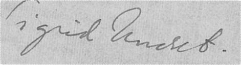Sigrid Undset.
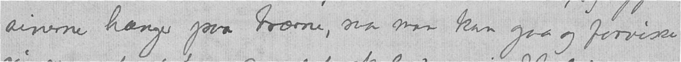sinerne hænger paa trærne, saa man kan gaa og forvisse
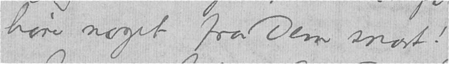høre noget fra Dem snart!
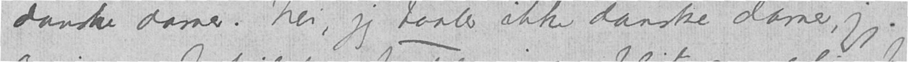danske damer. Nei, jeg taaler ikke danske damer, jeg.
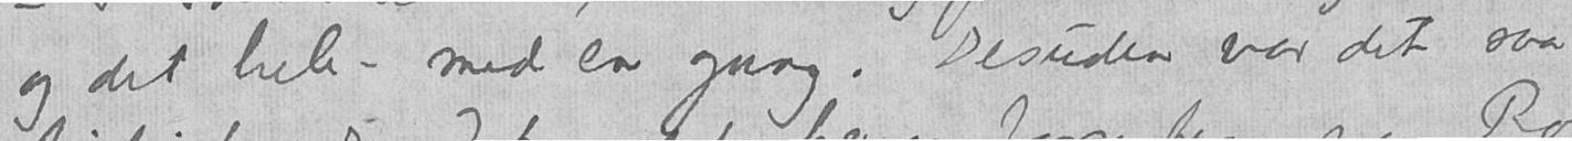og det hele – med en gang. Desuden var det saa
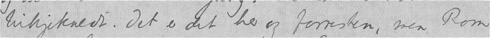bikjekaldt. Det er det her og forresten, men Rom
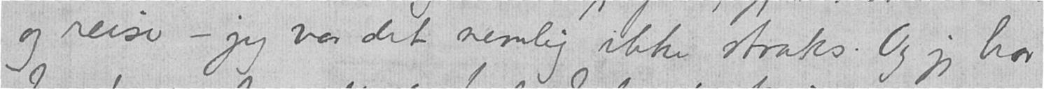og reise – jeg var det nemlig ikke straks. Og jeg har
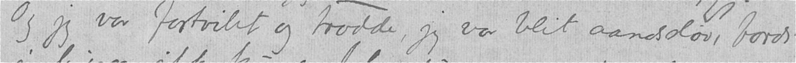Og jeg var fortvilet og trodde, jeg var blit aandsløv, fordi
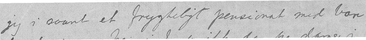jeg i saant et frygteligt pensionat med bare
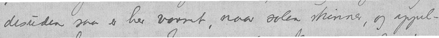desuden saa er her varmt, naar solen skinner, og appel-
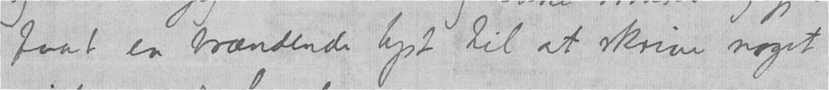faat en brændende lyst til at skrive noget
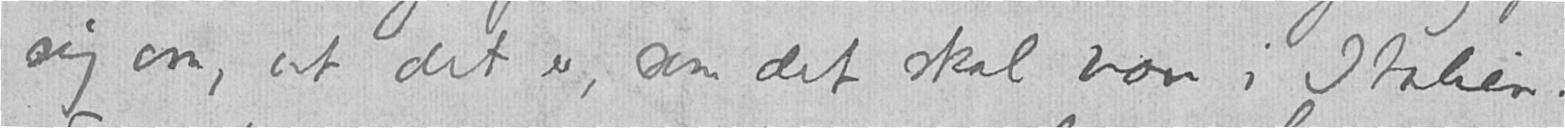sig om, at det er, som det skal være i Italien.
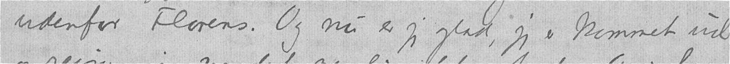udenfor Florens. Og nu er jeg glad, jeg er kommet ud
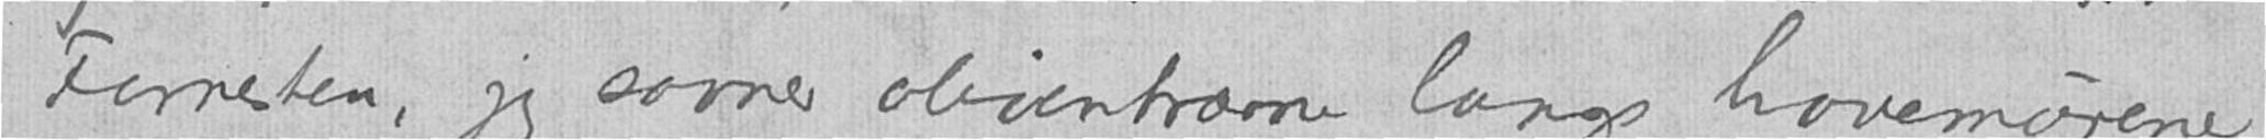Forresten, jeg savner oliventrærne langs havemurene
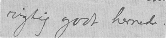rigtig godt hernede.
